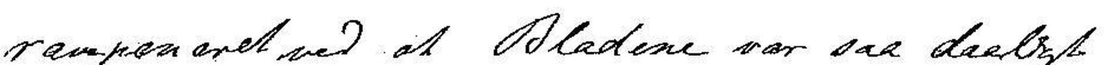ramponeret ved at Bladene var saa daarligt
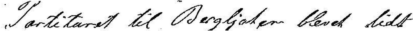Partituret til Bergljot er blevet lidt
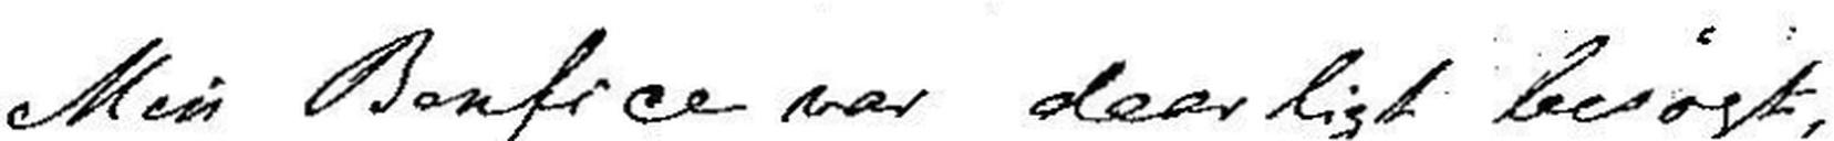Min Benfice var daarligt besøgt,
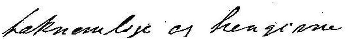taknemlige og hengivne
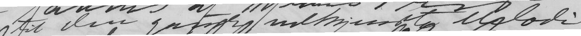til den ganske udkjendte Melodi.
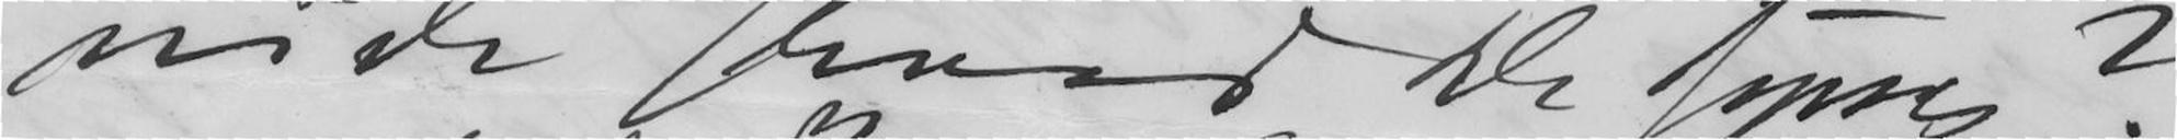vide hvad De synes?
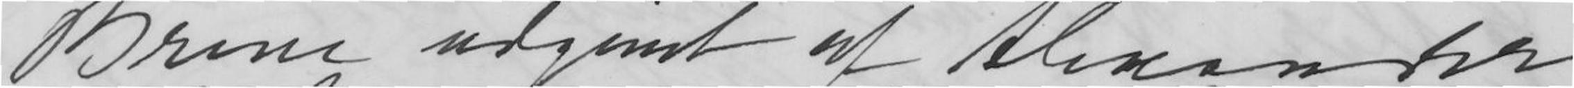Breve udgivet af Alexander
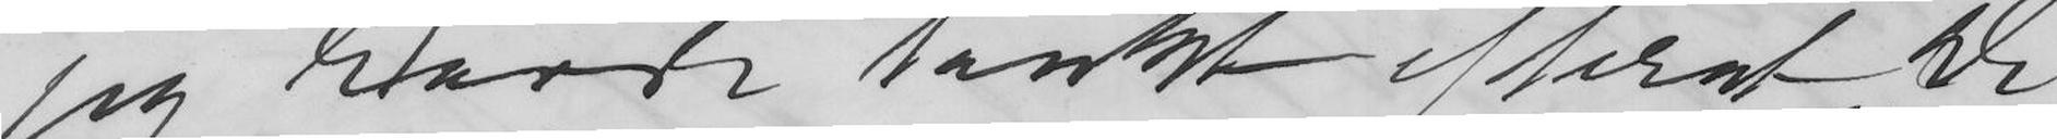jeg havde tænkt efterat De
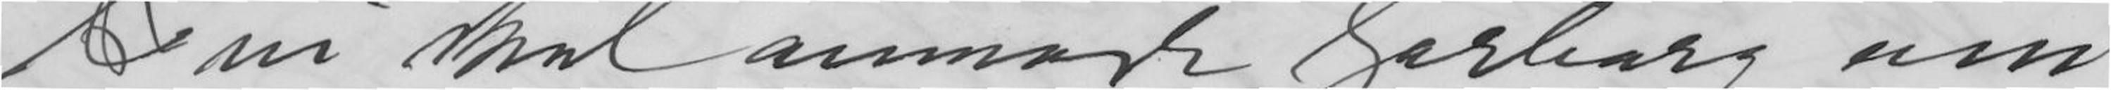l vi skal anmode Garborg om
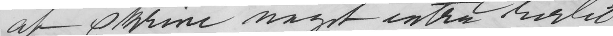at skrive noget extra hertil
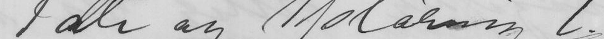Tale og Afsløring.
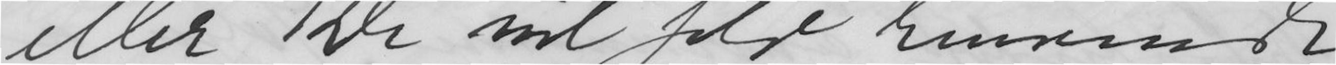eller De vil selv henvende
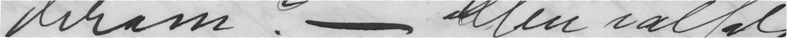derom? - Men ialfald
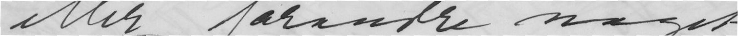eller forandre noget
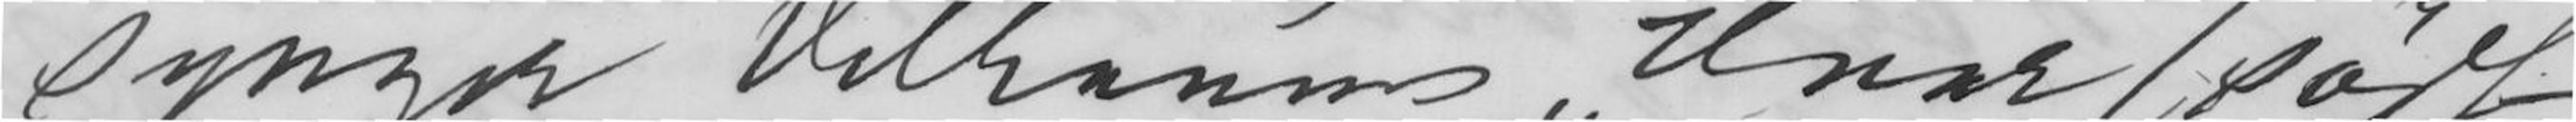synger Velhavens Hvor sødt
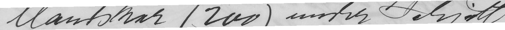1) Mandskor (200) under Schjøtt
